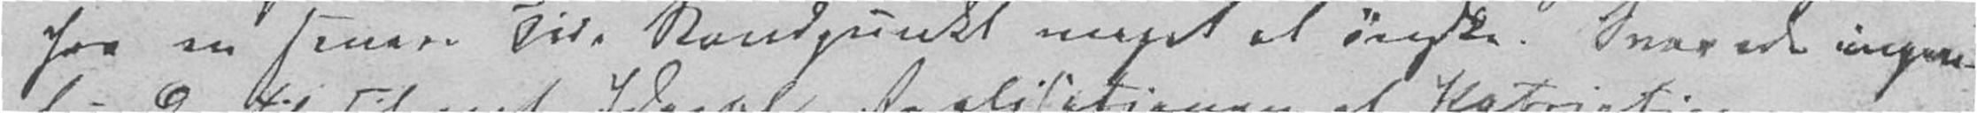fra en senere Tids Standpunkt meget at ønske. Svarede ingen-
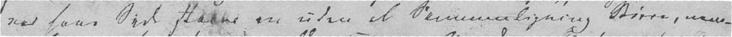ved hans Side staaer en uden al Sammenligning Større, nem-
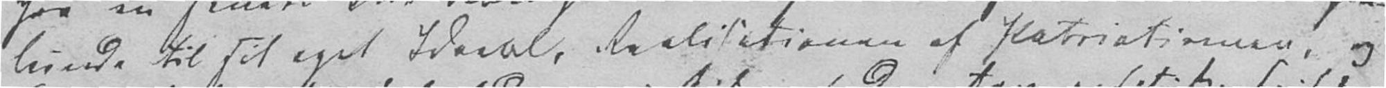lunde til sit eget Ideeal, Realisationen af Patriotismen, og
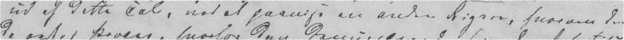ud af dette Tal, ved at paavise en anden Rigere, hvorom der
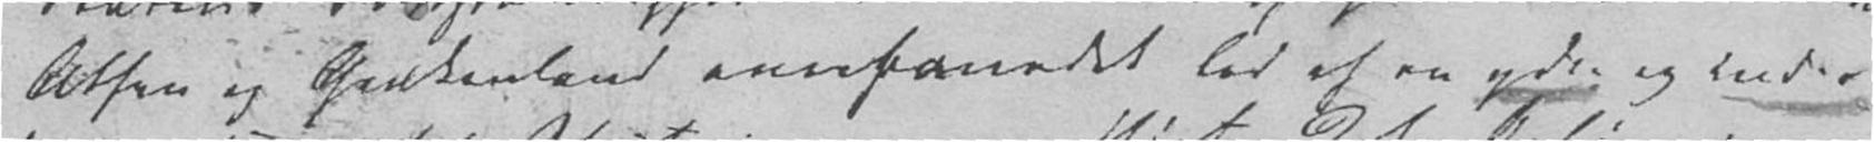Athen og Grækenland overhovedet led af en ydre og indre
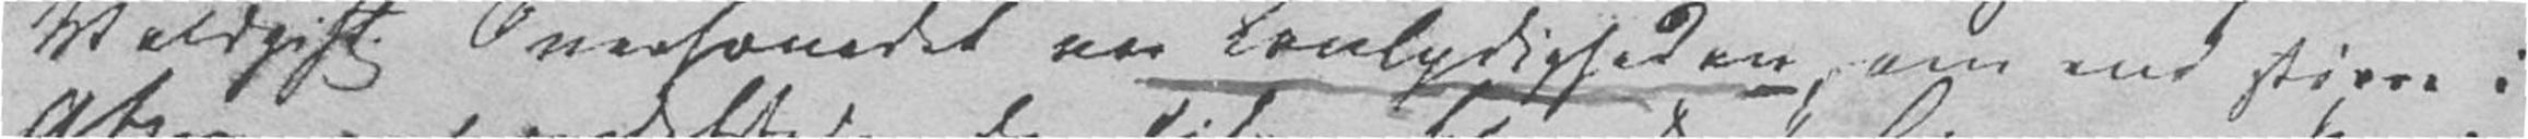Voldgift. Overhovedet var Lovlydigheden, om end større i
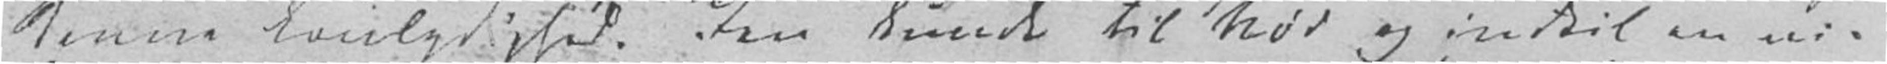denne Lovlydighed. Den kunde til Nød og indtil en vis
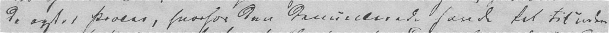da opstod Proces, hvorfor den denuencierede havde Ret til uden
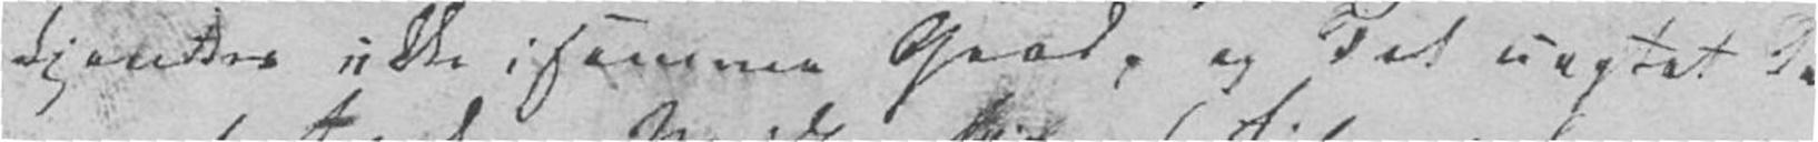kjendtes ikke i samme Grad, og det uagtet de
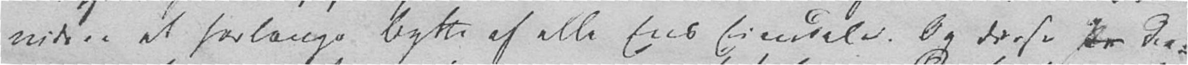videre at forlange Bytte af alle Ens Eiendele. Og disse De-
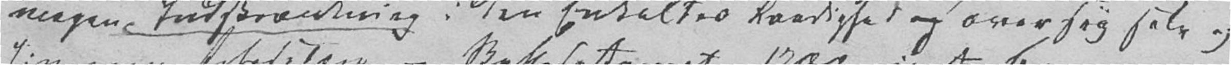megen Indskrænkning i den Enkeltes Raadighed og over sig selv og
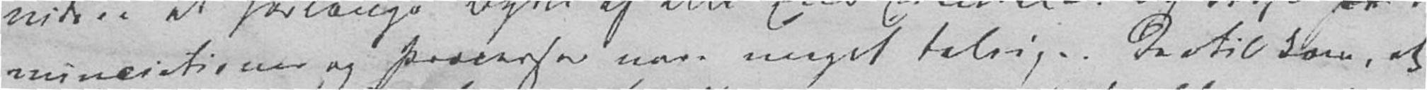nunciationer og Processe vare meget talrige. Dertil kom, at
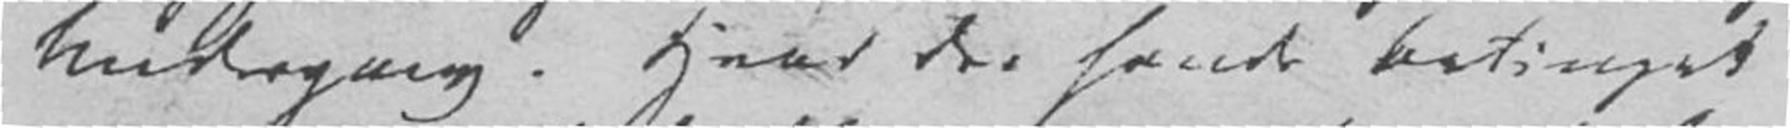Undergang. Hvad der havde betinget
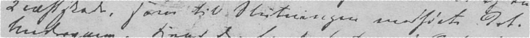Kræftskade, som til Slutningen medførte dets
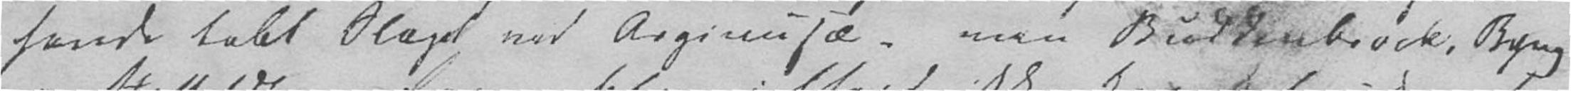havde tabt Slaget ved Orgivusæ, men Buddenbrock, Syng
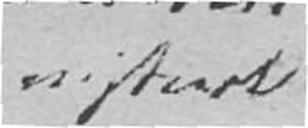vistnok
